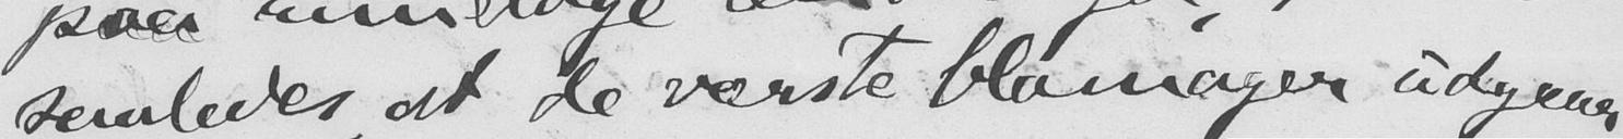saaledes, at de værste blamager udgaar,
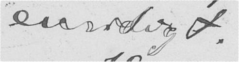ensidigt.
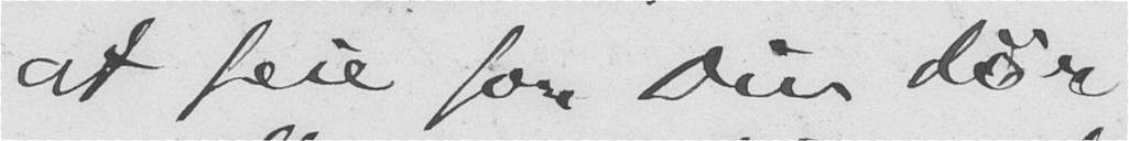at feie for Din dør.
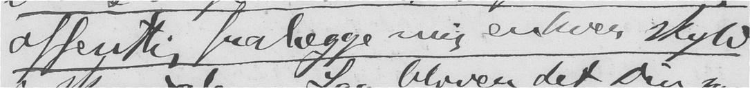offentlig fralægge mig enhver skyld
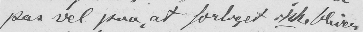pas vel paa, at forliget ikke bliver
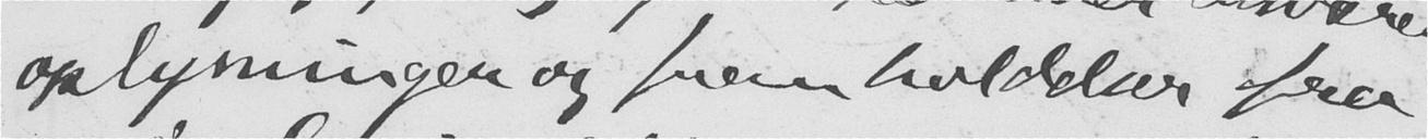oplysninger og fremholdelser fra
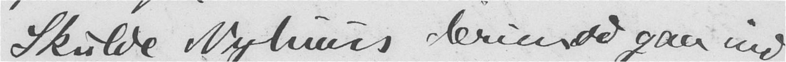Skulde Nyhuus derimod gaa ind
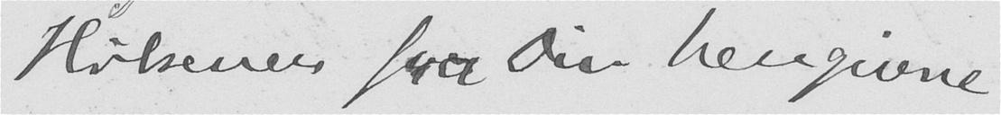Hilsener fra Din hengivne
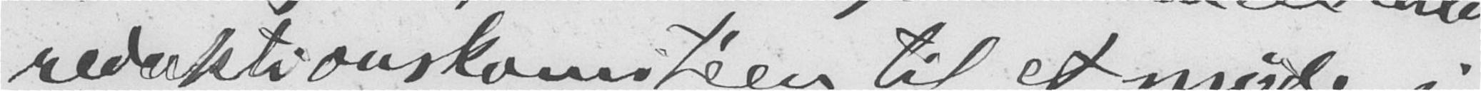redaktionskomitéen til et møde i
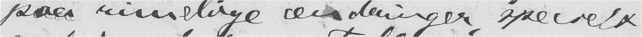paa rimelige ændringer, specielt
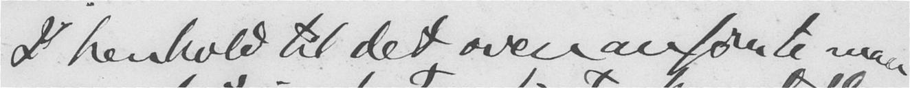I henhold til det overanførte maa
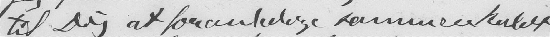til Dig at foranledige sammenkaldt
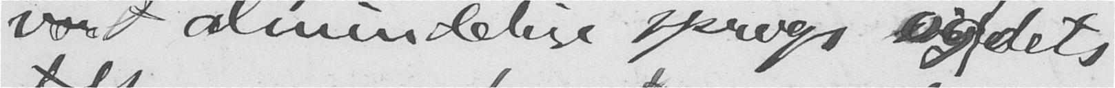vort almindelige sprogs og dets
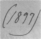(1897)
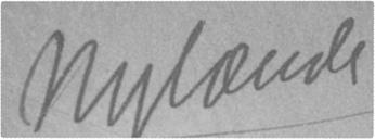Nylænde
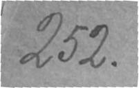252.
